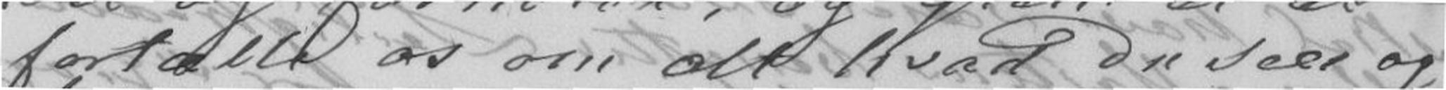fortælle os om alt hvad Du seer og
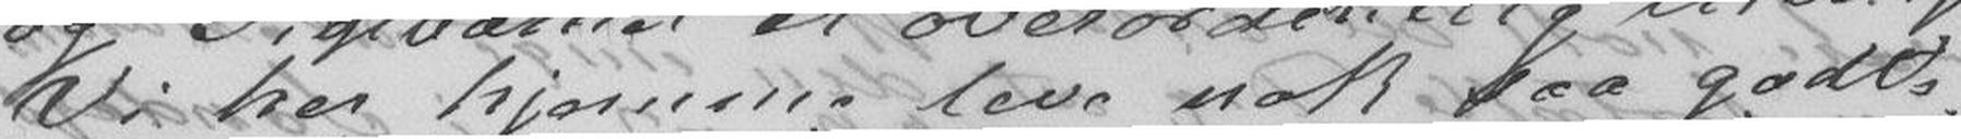Vi her hjemme leve nok saa godt,
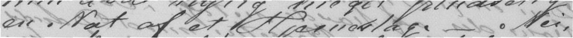en Nat af et Hjerneslag. - Nei,
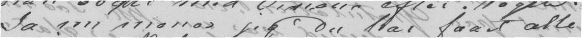Ja nu menes jeg Du her faaet alle
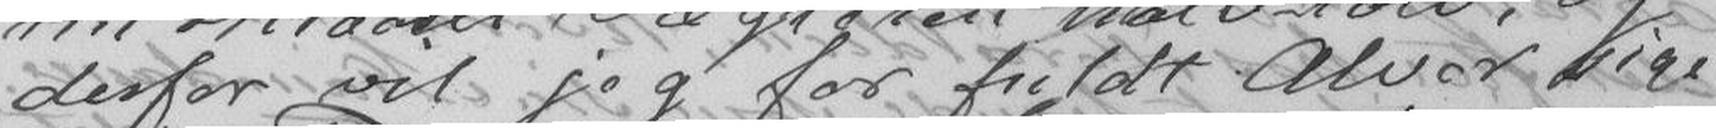derfor vil jeg for fuldt Alvor sige
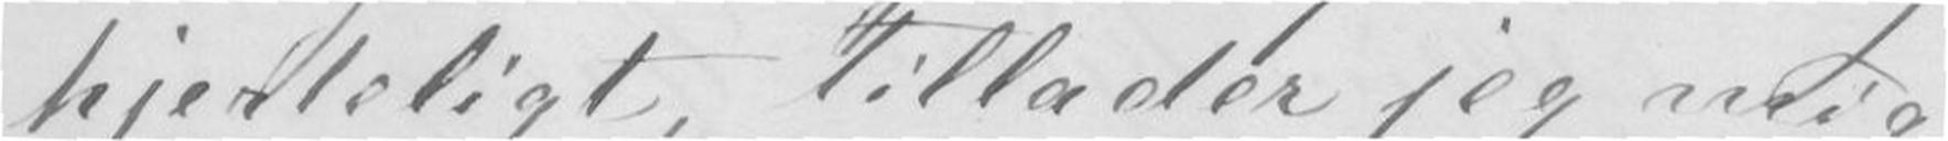hjerteligt, tillader jeg mig
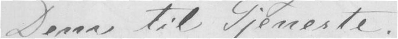Dem til Tjeneste.
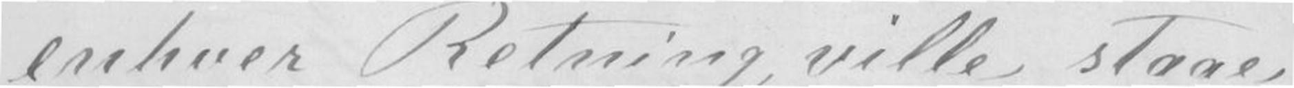enhver Retning, ville staae
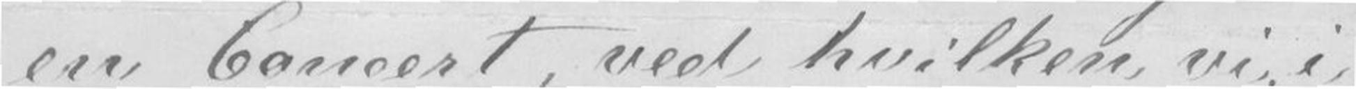en Concert, ved hvilken vi, i
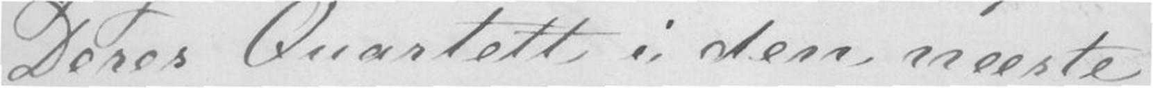Deres Quartett i den næste
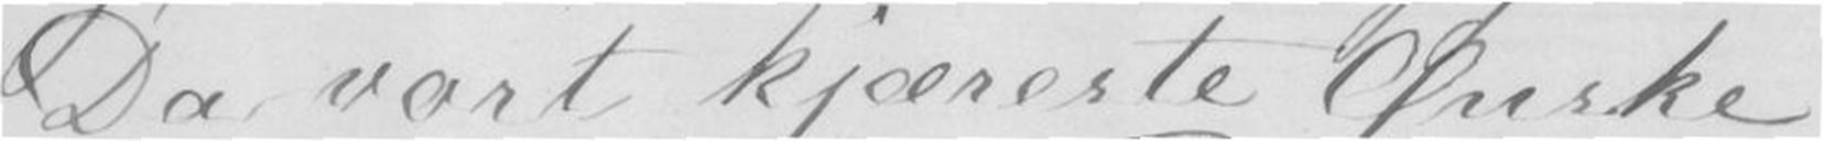Da vort kjæreste Ønske,
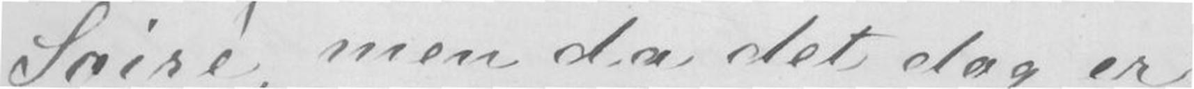Soiré, men da det dog er
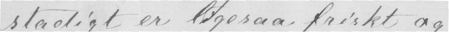stadig er ligesaa frisk og
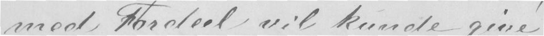med Fordeel vil kunde give
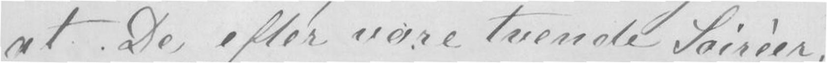at De efter vore tvende Soiréer,
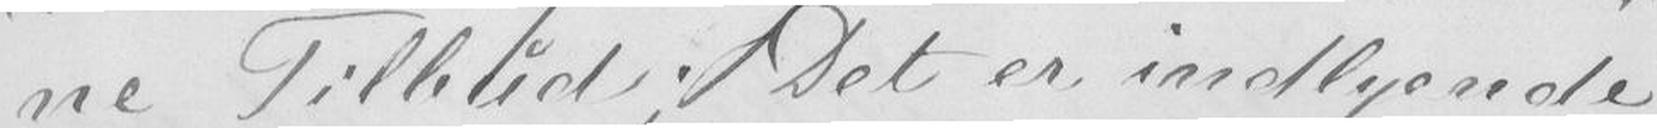ne Tilbud; Det er indlyende,
